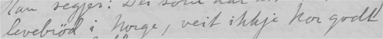levebröd i Norge, veit ikkje kor godt
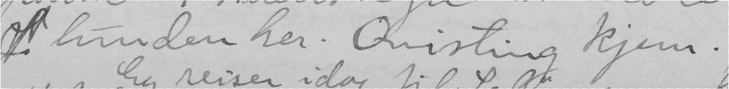lunden her. Qvisling kjem.
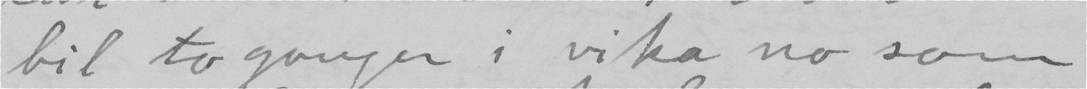bil to gonger i vika no som
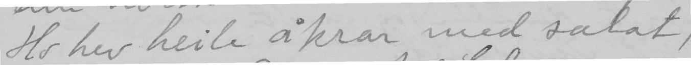Ho hev heile åkrar med salat,
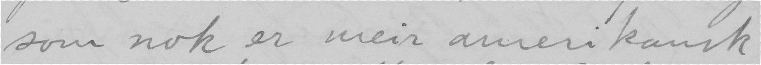som nok er meir amerikansk
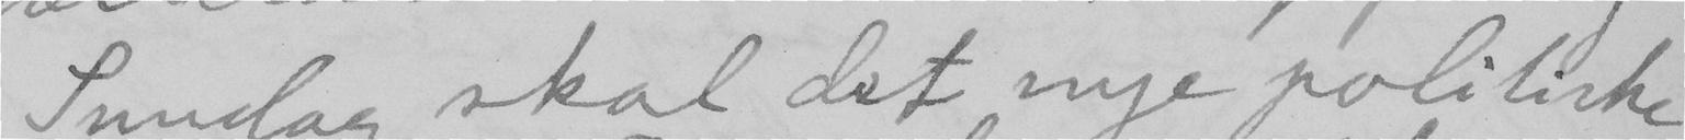Sundag skal det nye politiske
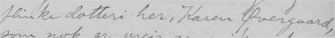flinke dotteri her, Karen Øvergaard,
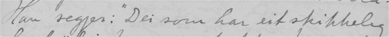Han segjer: "Dei som har eit skikkeleg
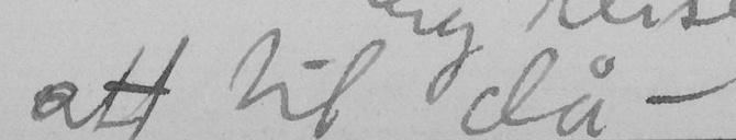att til då –
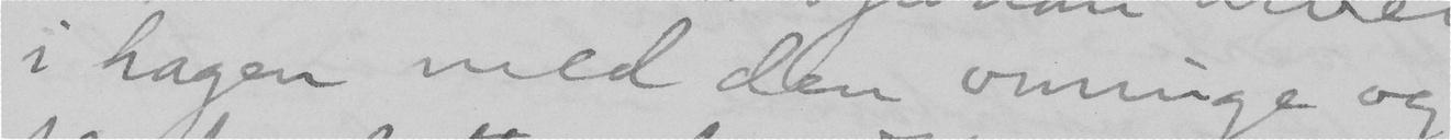i hagen med den omuge og
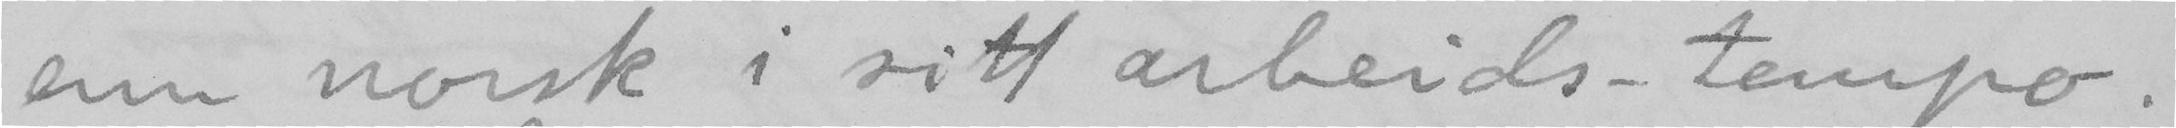enn norsk i sitt arbeids-tempo.
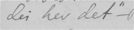dei her det" –
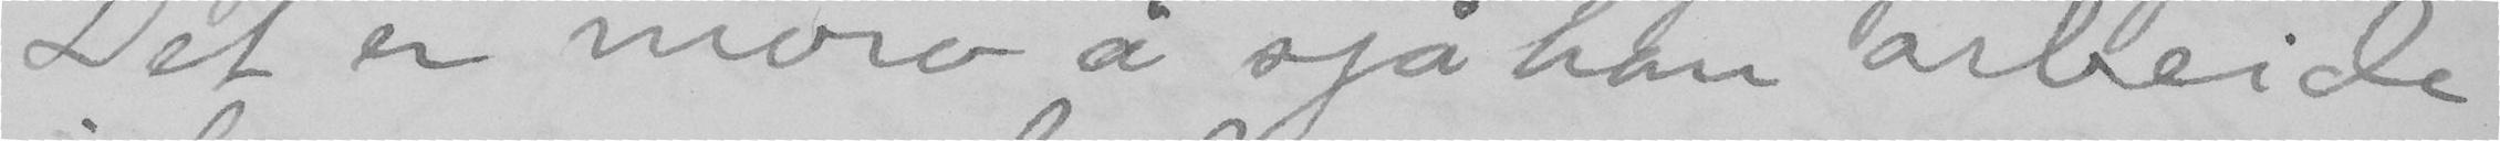Det er moro å sjå han arbeide
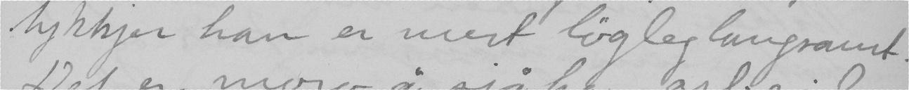tykkjer han er mest lögleg langsamt.
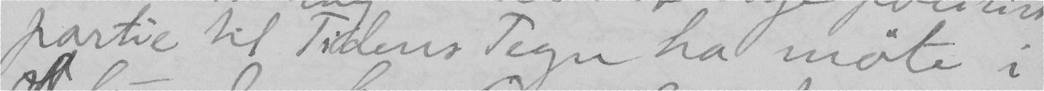partie til Tidens Tegn ha möøte i
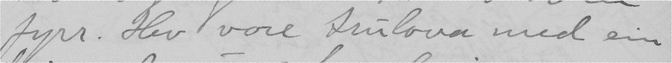fyrr. Hev vore trulova med ein
